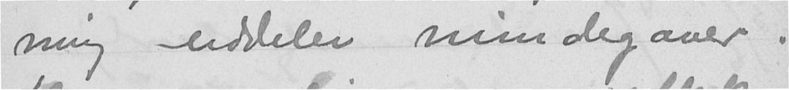ning uddeler mindegaver.
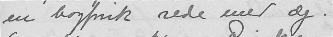en bogfink rede med æg.
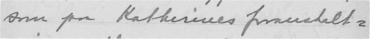som paa Kathrines foranstalt-
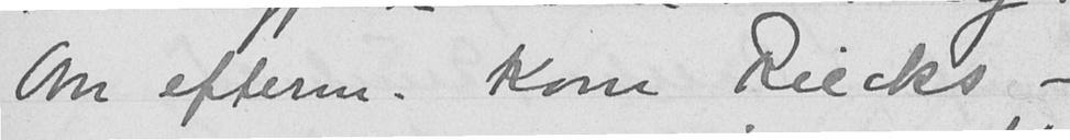Om efterm. kom Riecks -
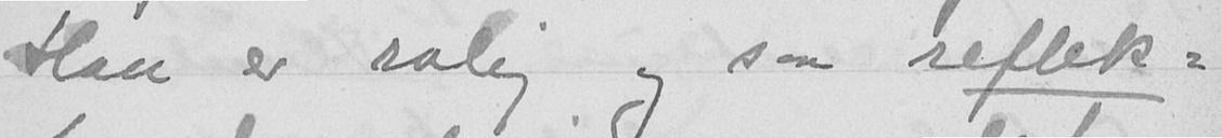Han er rolig og saa reflek-
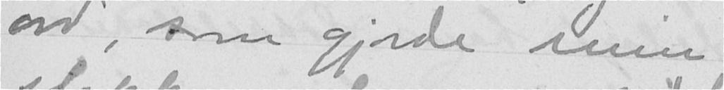ord, som gjorde min
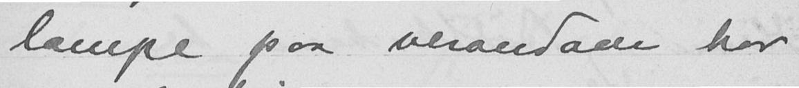lampe paa verandaen har
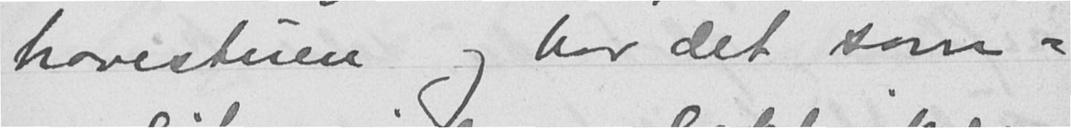hovestuen og har det som-
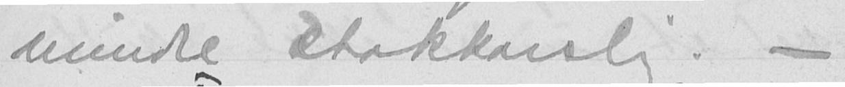mindre stakkarslig. -
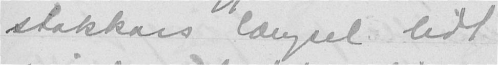stakkars længsel lidt
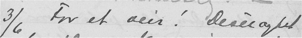3/6 For et veir! Desuagtet
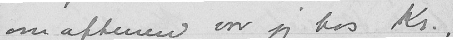om aftenen var jeg hos Kr.,
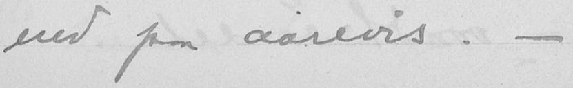end paa aarevis. -
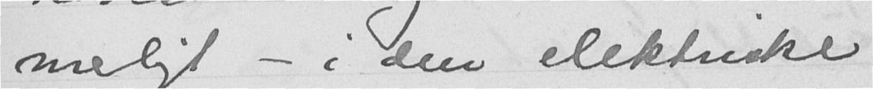merligt - i den elektriske
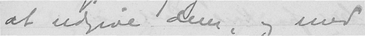at udgive dem, og med
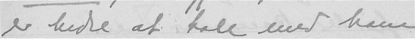er bedre at tale med ham
